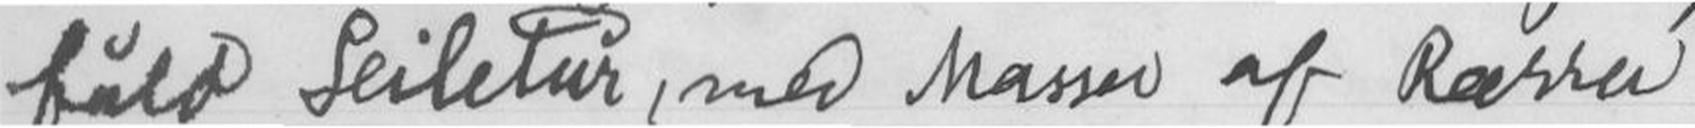fuld Seiletur, med Masser af Rækker
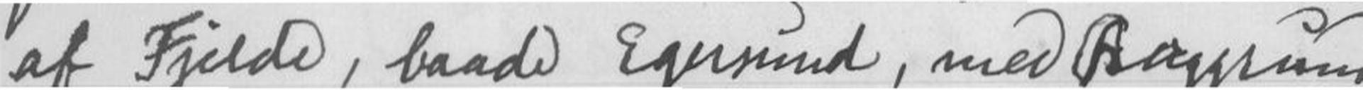af Fjelde, baade Egersund, med Baggrund
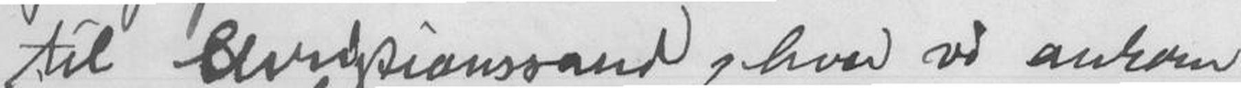til Christiansand, hvor vi ankom
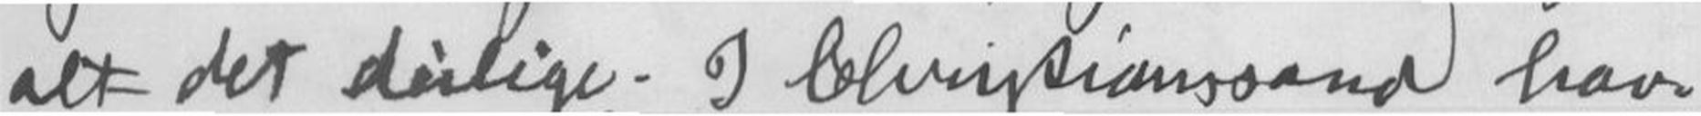alt det deilige. I Christiansand hav-
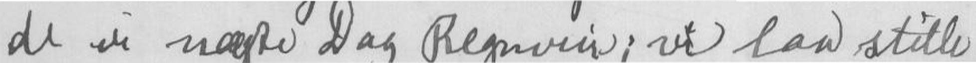de vi næste Dag Regnveir; vi laa stille
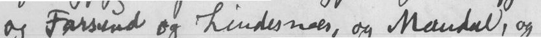og Farsund og Lindesnes, og Mandal, og
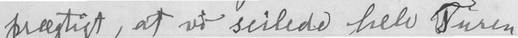prægtigt, at vi seilede hele Turen
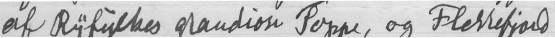af Ryfylkes grandiose Toppe, og Flekkefjord
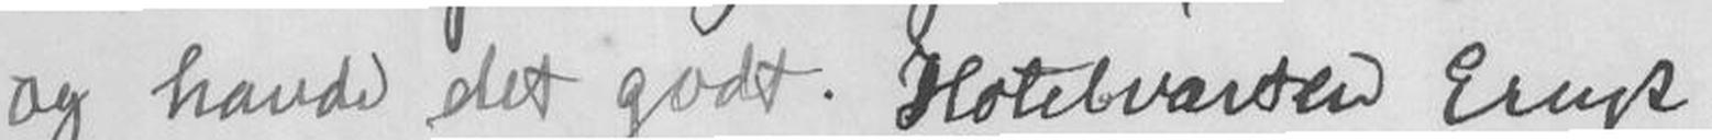og havde det godt. Hotelværten Ernst
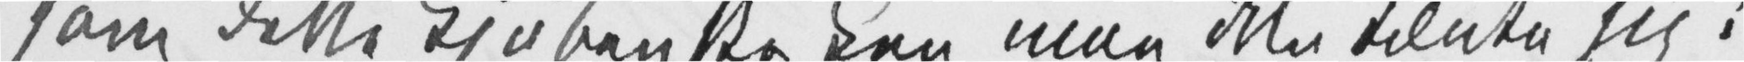som dette Kjøbenhavnske kan man ikke tænke sig!
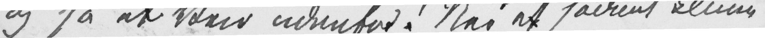og så et Veir udenfor! Nei et sådant Klima
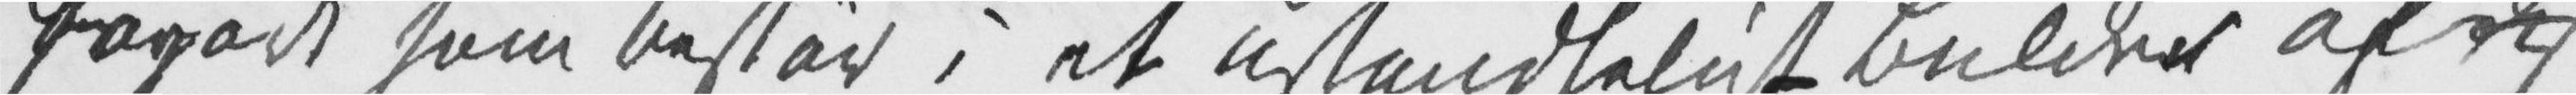sågodt som består i et ustandseligt Bulder af dis
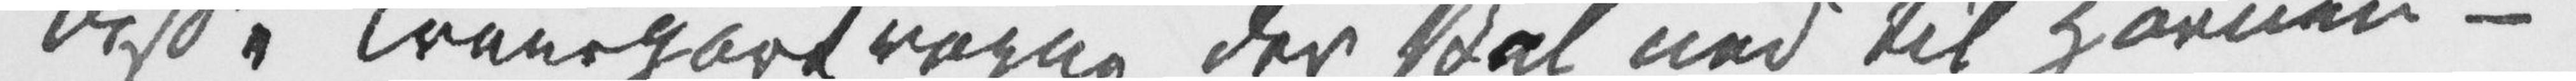disse Transportvogne der skal ned til Havnen –
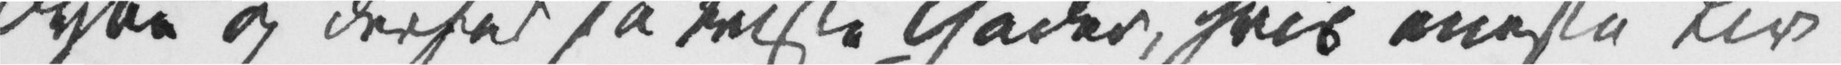dybe og derfor så triste Gader, hvis eneste Liv
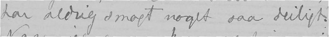har aldrig smagt noget saa deiligt.
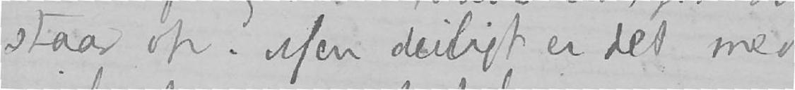staar op. Men deiligt er det med
